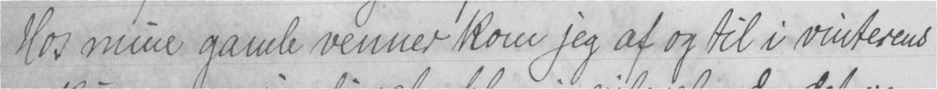Hos mine gamle venner kom jeg af og til i vinterens
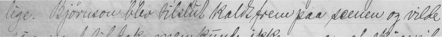lige. Bjørnson blev tilslut kaldt frem paa scenen og vilde
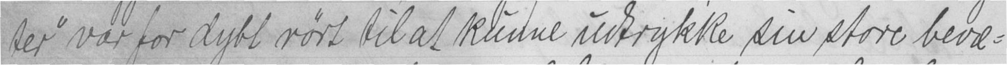ter" var for dybt rørt til at kunne udtrykke sin store bevæ-
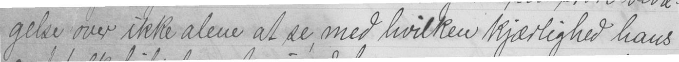gelse over ikke alene at se, med hvilken kjærlighed hans
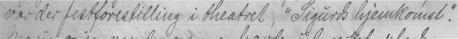var der festforestilling i theatret, "Sigurds hjemkomst."
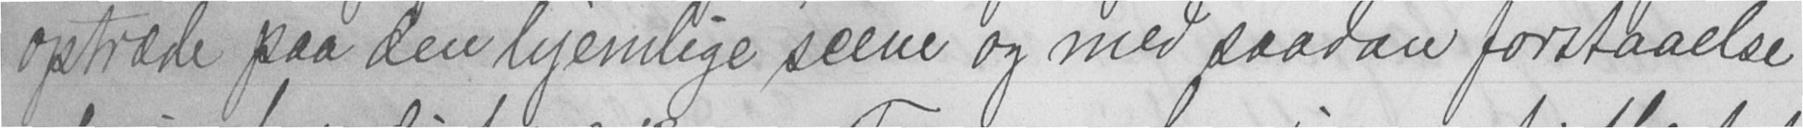optræde paa den hjemlige scene og med saadan forstaaelse
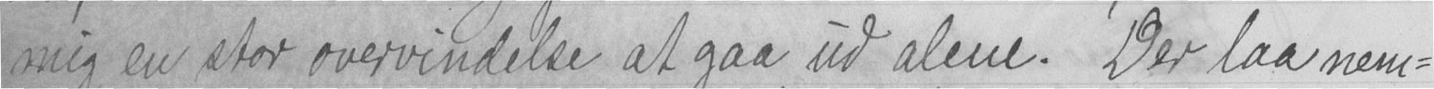mig en stor overvindelse at gaa ud alene. Der laa nem-
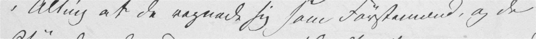i Alting at de regnede sig som Førstemænd, og der
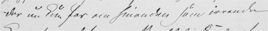der nu kun for om hinanden som irrende
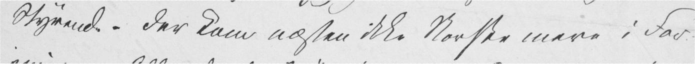Styrende – Der kom næsten ikke Norske mere i For-
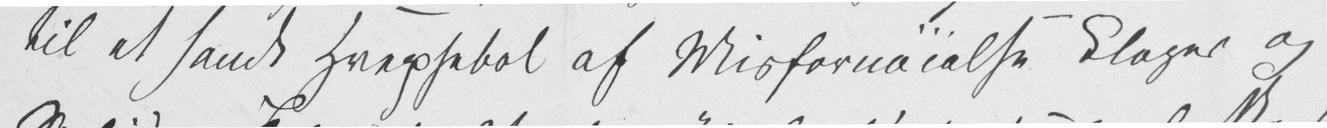til et sandt Hvepsebol af Misfornøielse Klager og
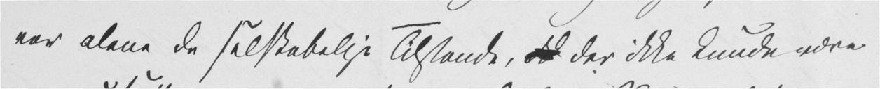var alene de selskabelige Tilstande, det der ikke kunde være
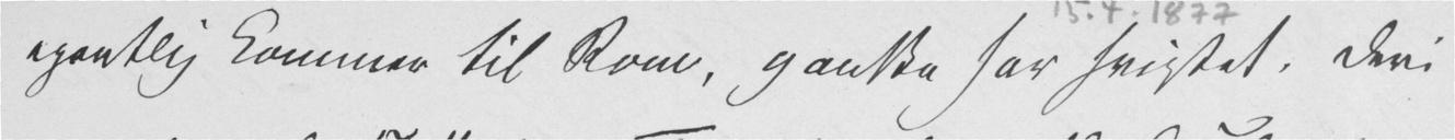egentlig kommer til Rom, ganske har svigtet, Deri
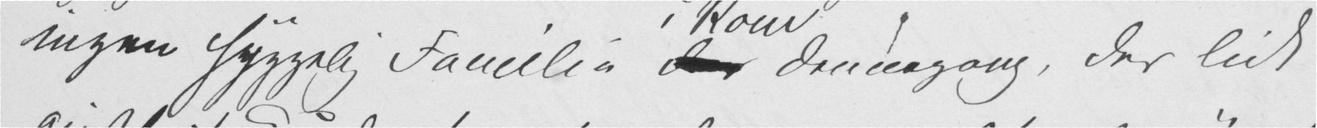ingen hyggelig Familie der dennegang, der lidt
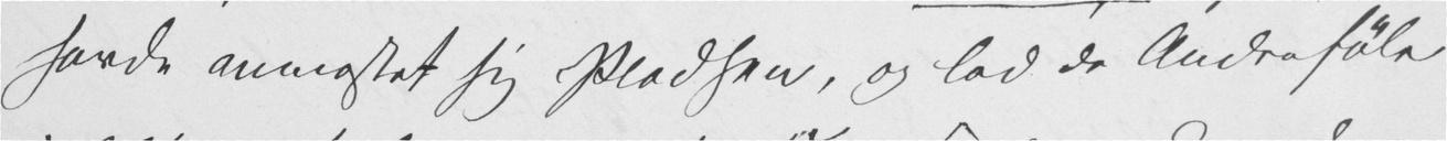havde anmæstet sig Pladsen, og lad de Andre føle
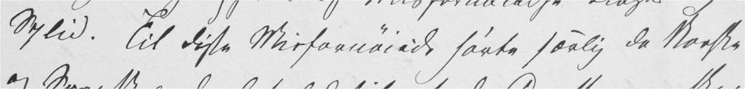Splid. Til disse Misfornøiede hørte særlig de Norske
